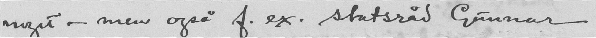noget - men også f. ex. statsråd Grunnar
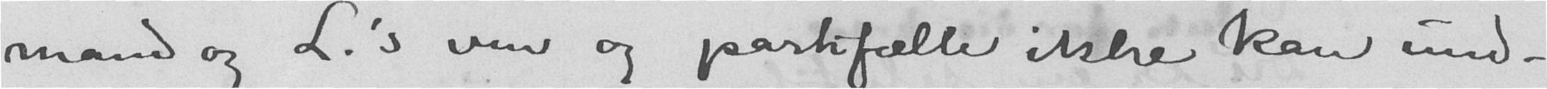mand og L.'s  ven og partifælle ikke kan und-
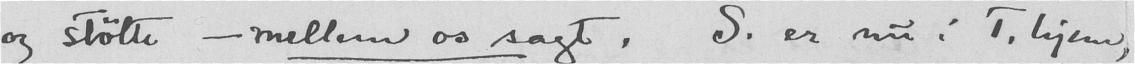og støtte - mellem os sagt. S. er nu i T.hjem,
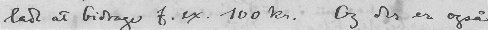lade at bidrage f .ex. 100 kr. Og der er også
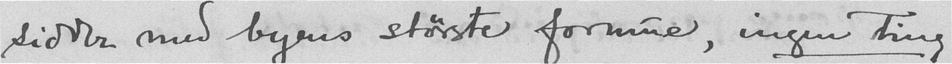sidder med byens største formue, ingen ting
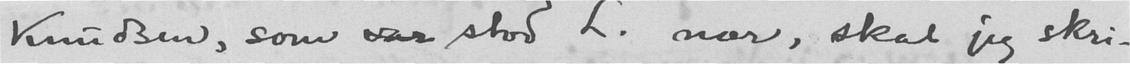Knudsen, som stod L. nær, sjak heg skri-
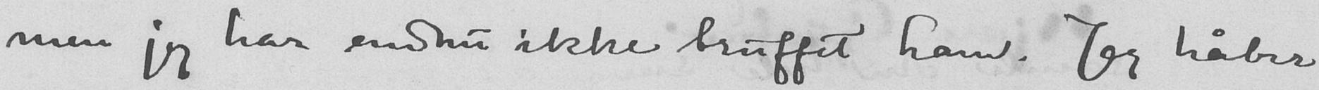men jeg har endnu ikke truffet ham. Jeg håber
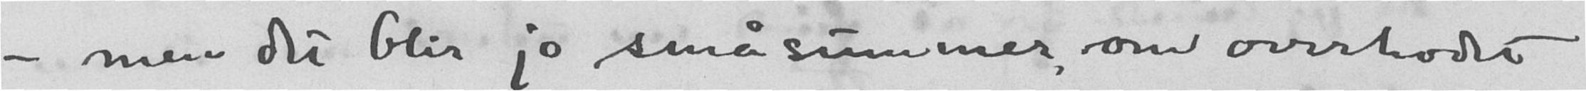- men det blir jo småsummer, om overhodet
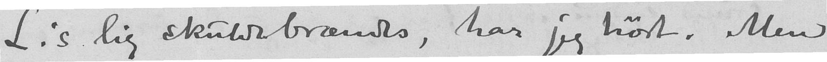L.'s lig skulde brændes, har jeg hørt. Men
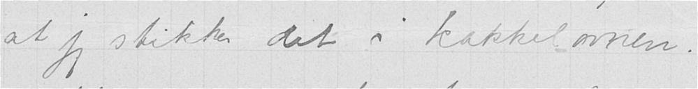at jeg stikker det i kakkelovnen.
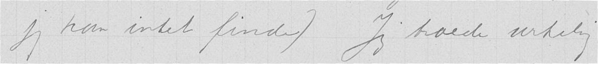jeg kan intet finde) Jeg troede virkelig
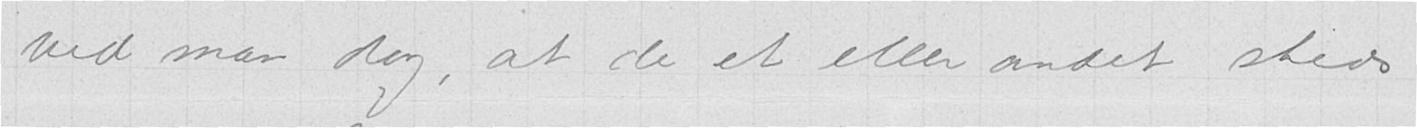ved man dog, at de et eller andet steds
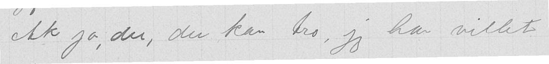Ak ja, du, du kan tro, jeg har villet
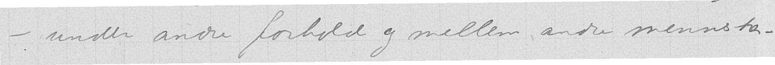- under andre forhold og mellem andre mennesker
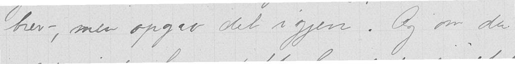brev, men opgav det igjen. Og om du
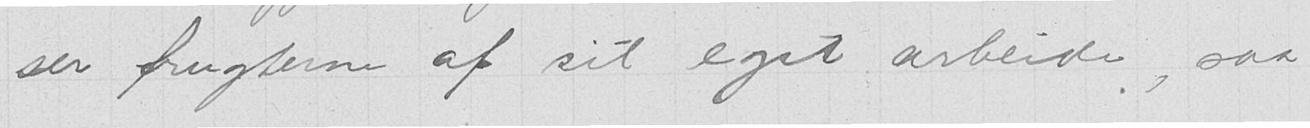ser frugterne af sit eget arbeide, saa
